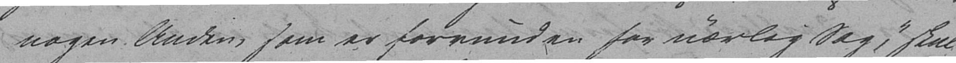nogen Anden, som er forvunden for uærlig Sag," skul-
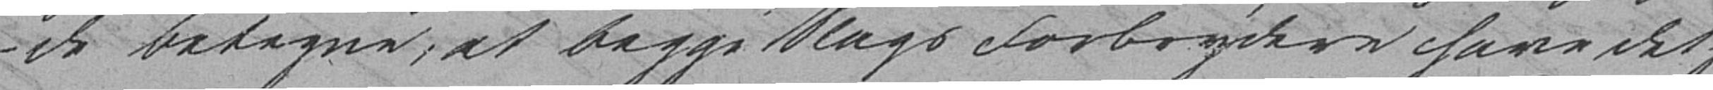de betegne, at begge Slags Forbrydere have det
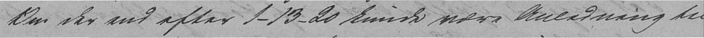Om der end efter 1-13-20 kunde være Anledning til
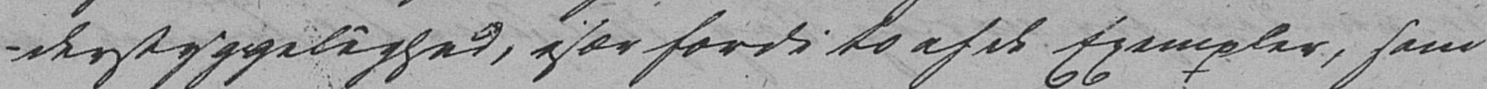derstyggelighed, især fordi to af de Exempler, som
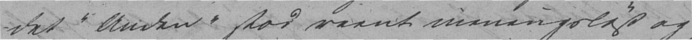det "Anden" stod reent meningsløst og
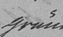Grund
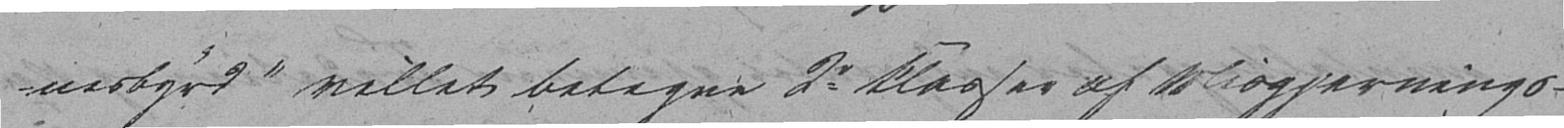nesbyrd" villet betegne 2e Classer af Misgjernings-
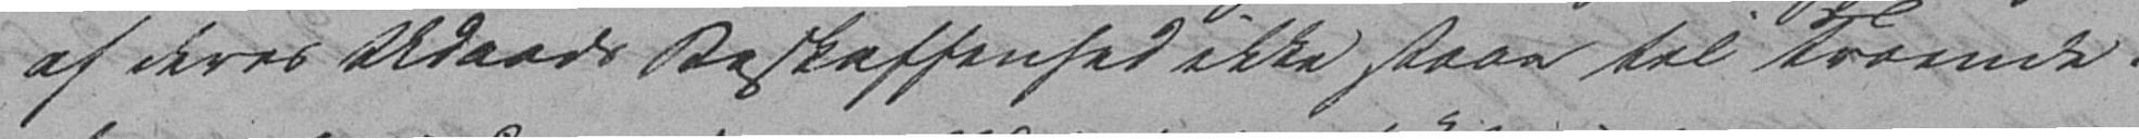af deres Udaads Beskaffenhed ikke staar til Troende.
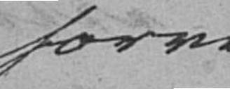forvir-
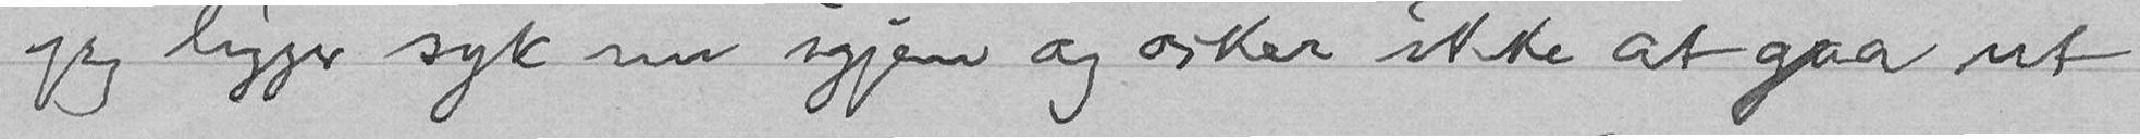jeg ligger syk nu igjen og orker ikke at gaa ut
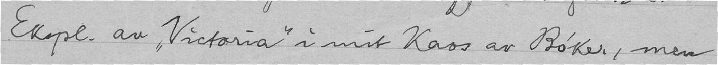Ekspl. av "Victoria" i mit Kaos av Bøker, men
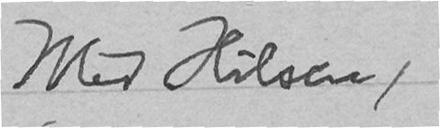Med Hilsen,
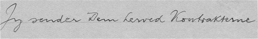Jeg sender Dem herved Kontrakterne
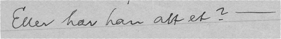Eller har han alt et? -
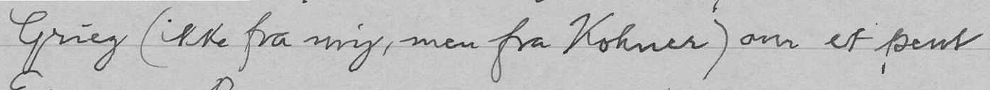Grieg (ikke fra mig, men fra Kohner) om et pent
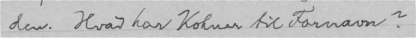den. Hvad har Kohner til Fornavn?
